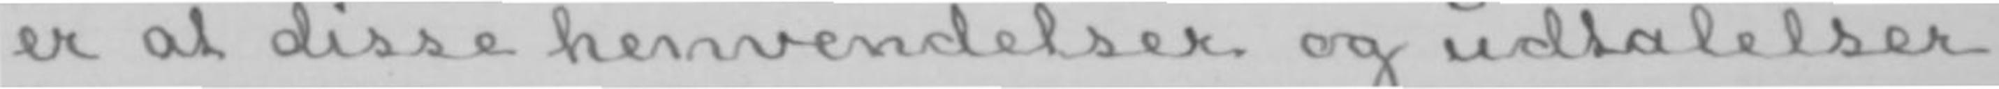er at disse henvendelser og udtalelser
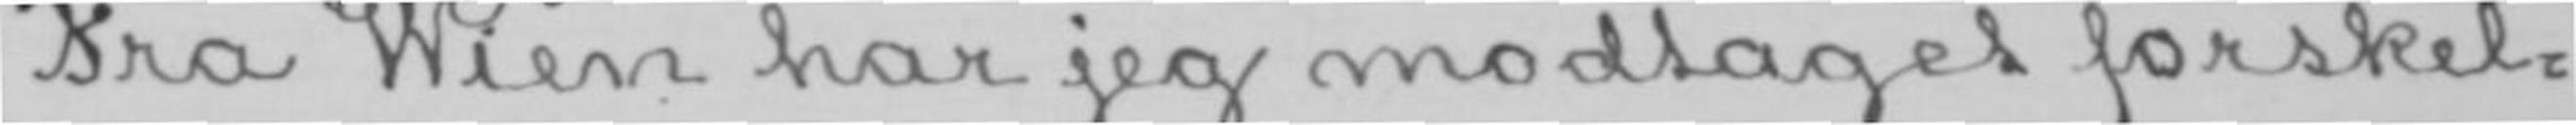Fra Wien har jeg modtaget forskel-
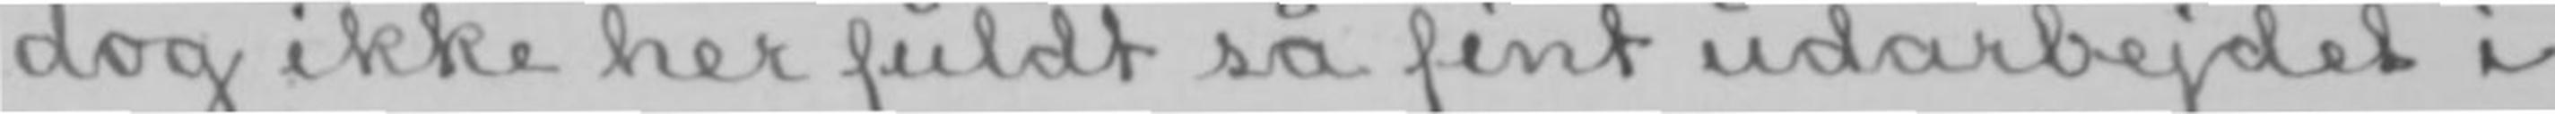dog ikke her fuldt så fint udarbejdet i
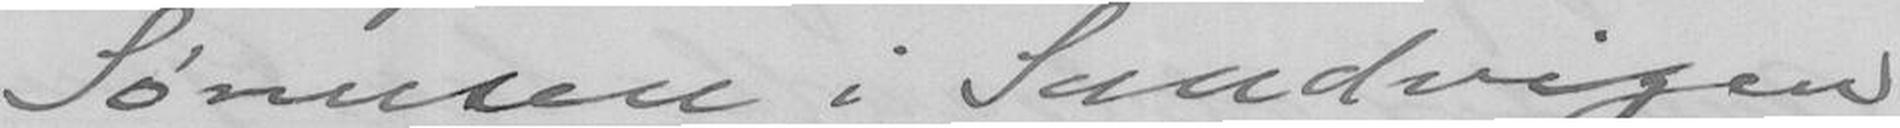Sørensen i Sandvigen
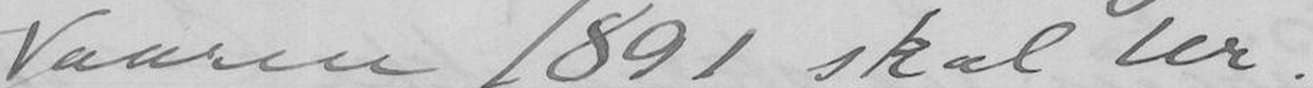Vaaren 1891 skal Hr.
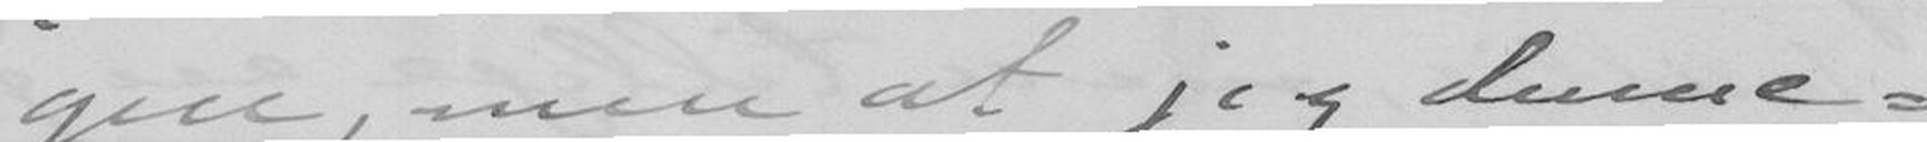gen, men at jeg denne
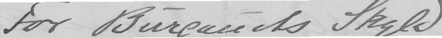For Burcauets Skyld
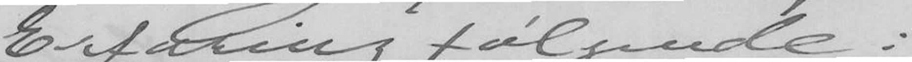Erfaring følgende:
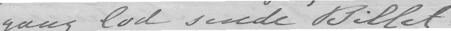gang lod sende Billet-
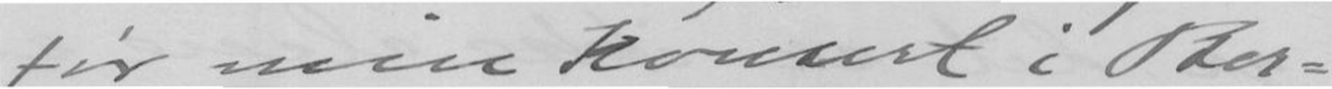før min Koncert i Ber-
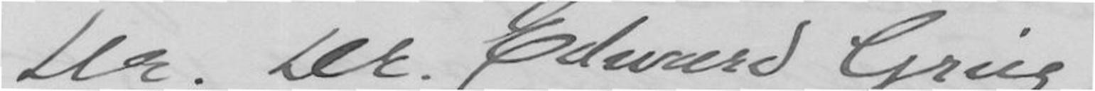Hr. Dr. Edward Grieg!
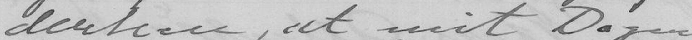derhen, at mit Dagen
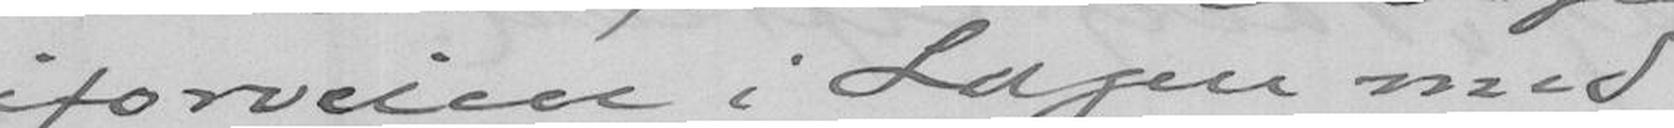iforveien i Sagen med
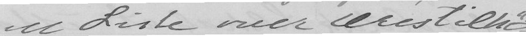en Liste med Ærestilhø-
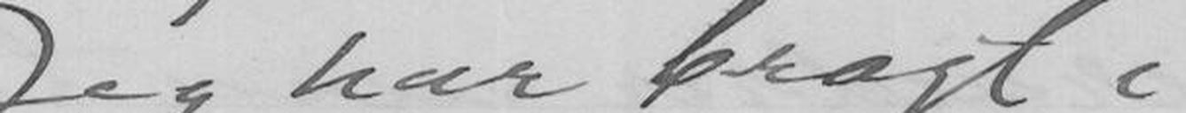Jeg har bragt i
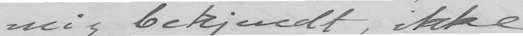mig bekjendt, ikke
